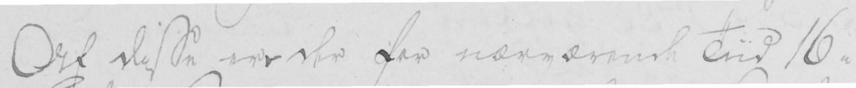Af disse er der for nærværende Tiid 16
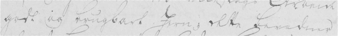godt og brugbart Jern; dette bevidner
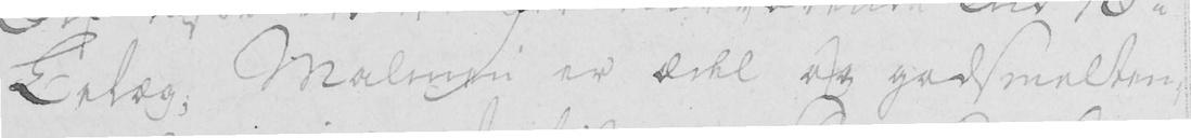Belæg; Malmen er ædel og godsmelten-
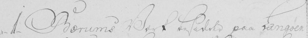"-1- Bærums Værk besidder paa Langøen
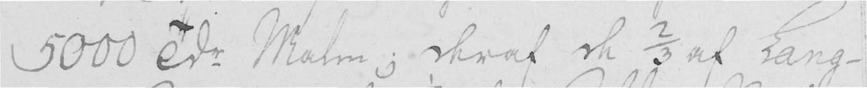5000 Tdr Malm; deraf de 2/3 af Lang-
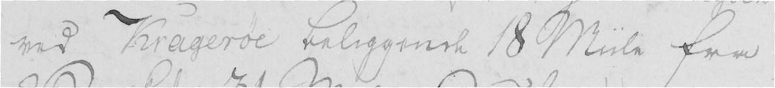ved Kragerøe beliggende 18 Miile fra
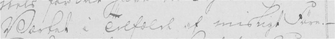Værket i Tilfælde af misligt Føre. -
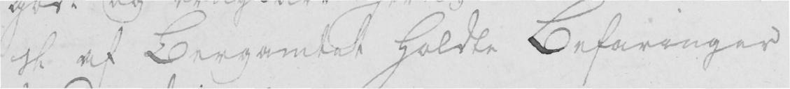de af Bergamtet holdte Befaringer
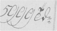5999 Tdr
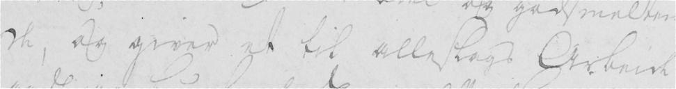de, og giver et til alleslags Arbeide
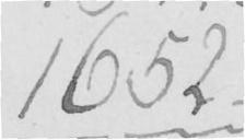1652
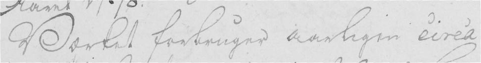Værket forbruger aarligen circa
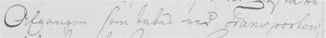Afgangen som tabes ved Transporten,
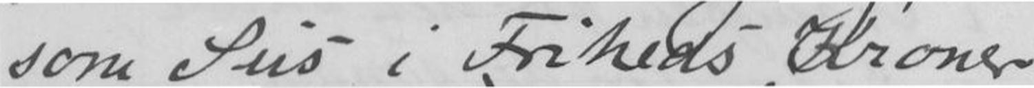som Sus i Friheds Kroner
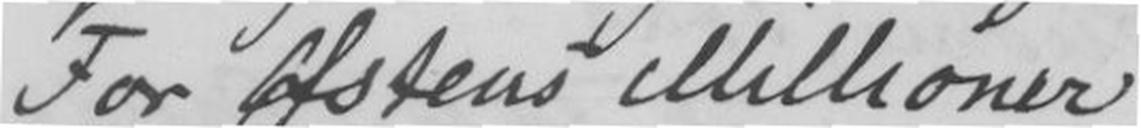For Østens Millioner
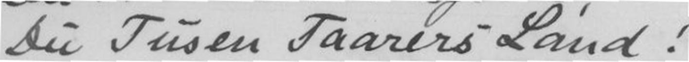Du Tusen Taarers Land!
